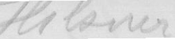Hilsner.
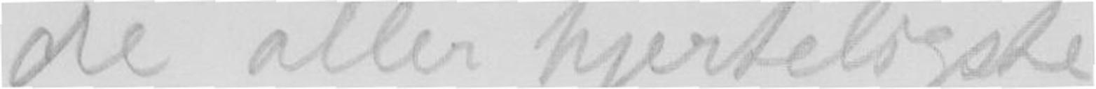de aller hjerteligste
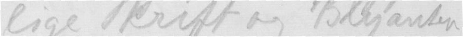lige Skrift og Blyanten.
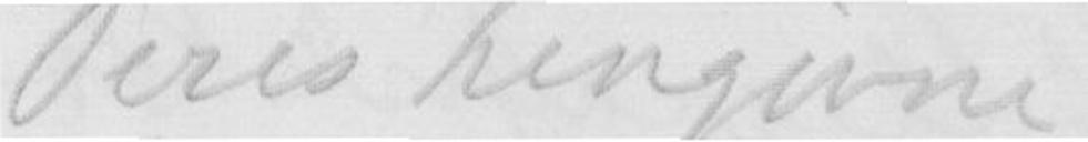Deres hengivne
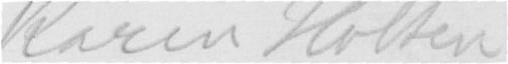Karen Holten
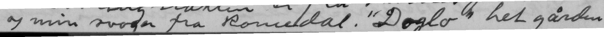og min svoger fra Romedal. "Doglo" het gården
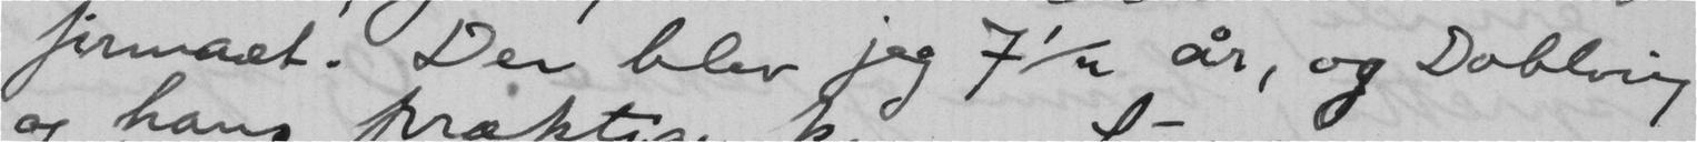firmaet. Der blev jeg 7½ år, og Dobloug
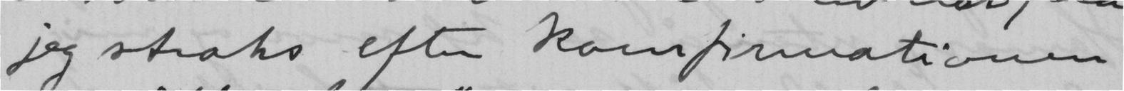jeg straks efter konfirmationen
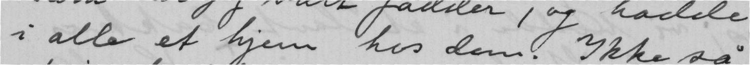i alle et hjem hos dem. Ikke så
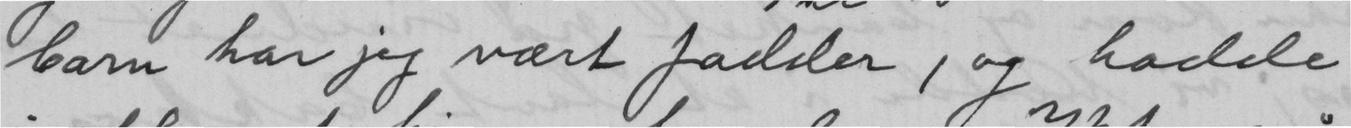barn har jeg vært fadder, og hadde
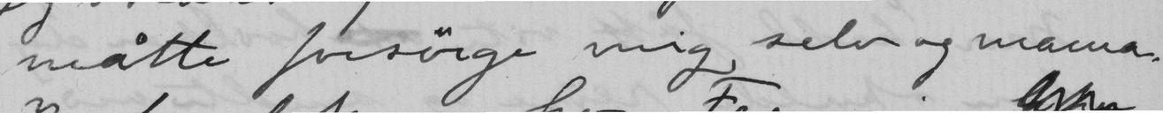måtte forsørge mig selv og mama.
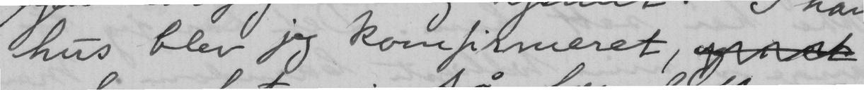hus blev jeg komfirmeret,
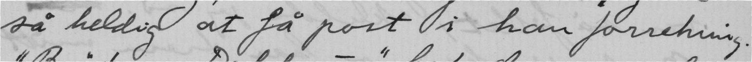så heldig at få post i hans forretning.
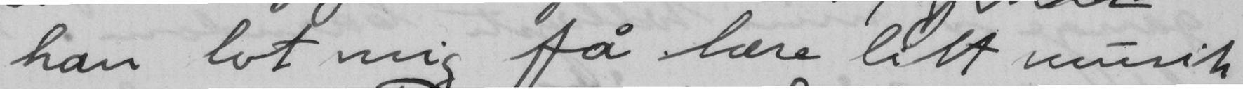han lot mig fa lære litt musik
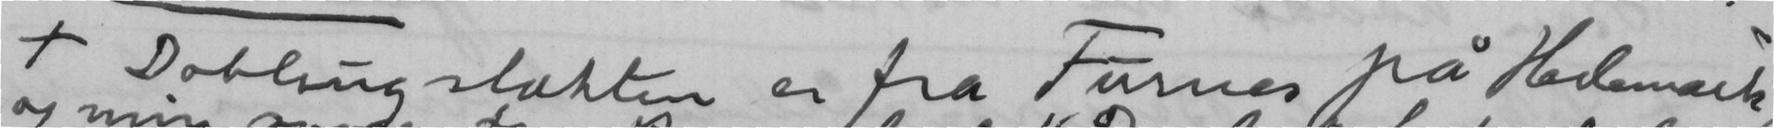x Doblougslækten er fra Furnes på Hedemark
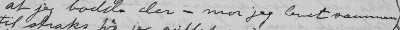at jeg bodde der - mor jeg levet sammen
